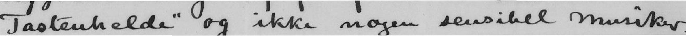Tastenhelde og ikke nogen sensibel musiker.
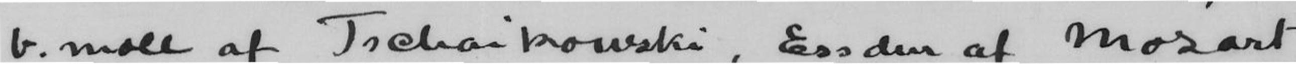b.moll af Tschaikowski, Essdur af Mozart
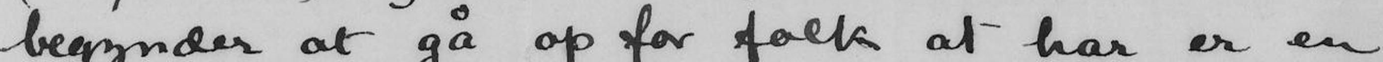begynder at gå op for folk at han er en
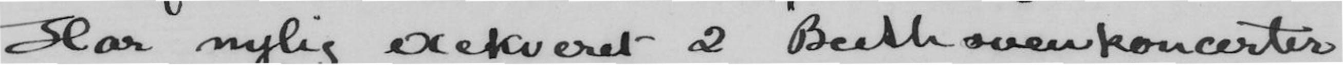Har nylig exekveret 2 Beethovenkoncerter
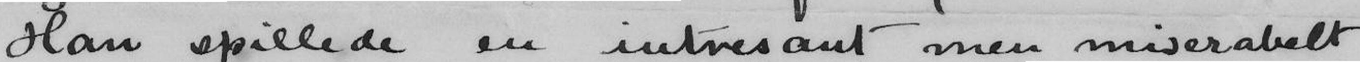Han spillede en intresant men miserabelt
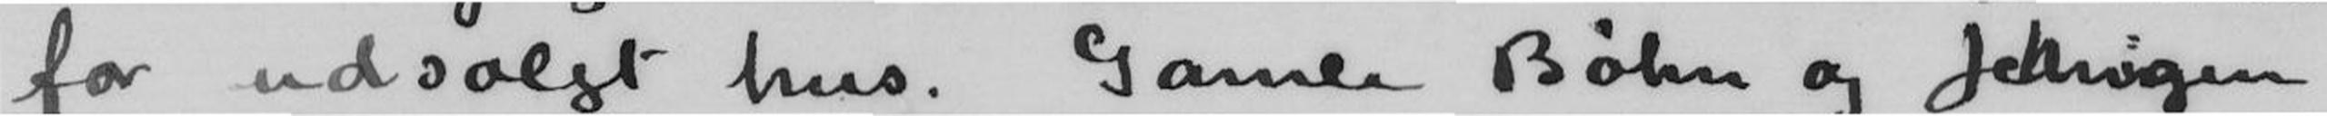for udsolgt hus. Gamle Bøhn og Schrøger
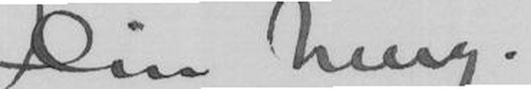Din heng.
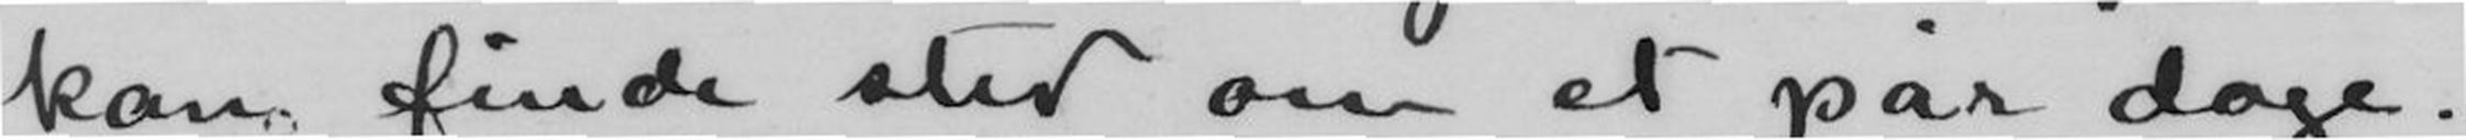kan finde sted om et par dage.
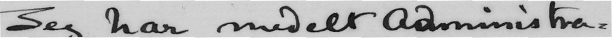Jeg har medelt Administra-
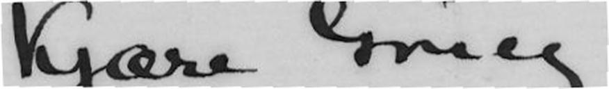Kjære Grieg!
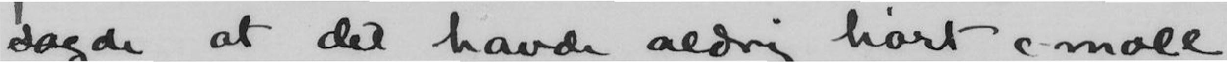sagde at de havde aldrig hørt c-moll
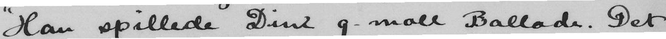han spillede Din g-moll Ballade. Det
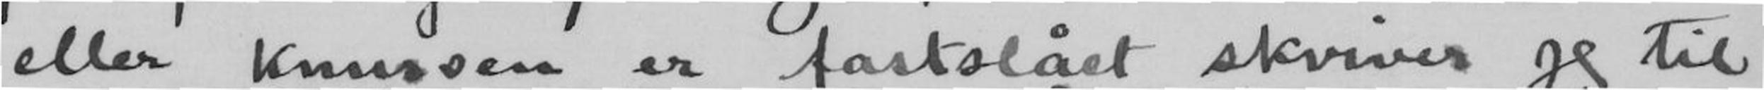eller Knussen er fastslået skriver jeg til
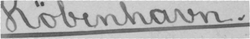København.
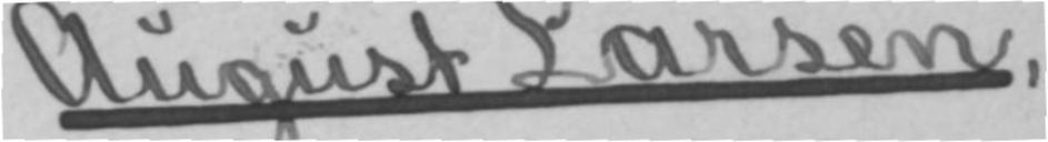August Larsen,
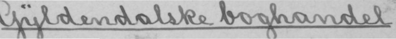Gyldendalske boghandel,
